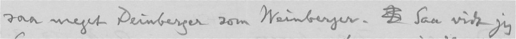saa meget Deinberger som Weinberger. L Saa vidt jeg
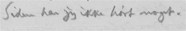Siden har jeg ikke hørt noget.
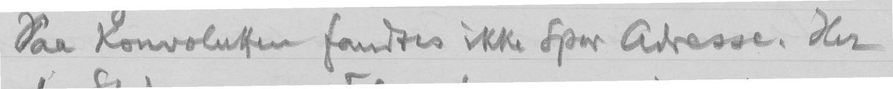Paa Konvolutten fandtes ikke Spor Adresse. Her
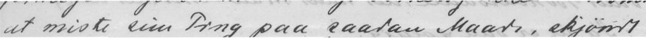at miste sine Ting paa saadan Maade, skjøndt
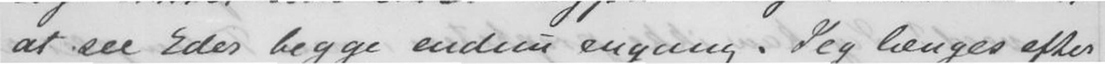at see Eder begge endnu engeng. Jeg længess efter
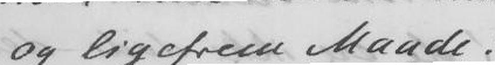og ligefrem Maade.
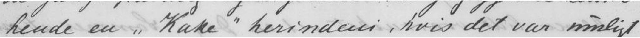hende en "Kake" herindeni, hvis det var muligt
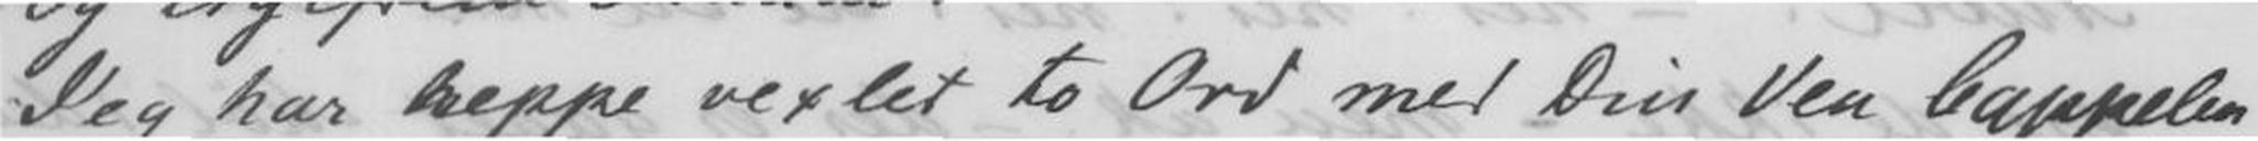Jeg har neppe vexlet to Ord med Din Ven Cappelen
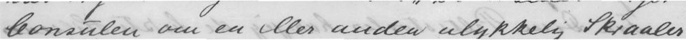Consulen om en eller anden ulykkelig Skraaler
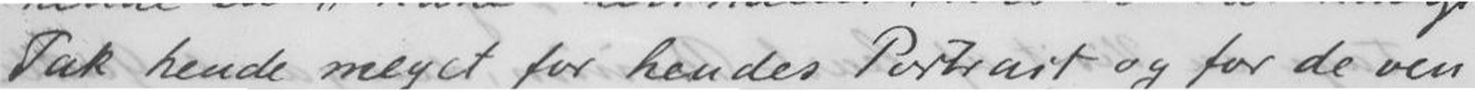Tak hende for handes Portrait og for de ven-
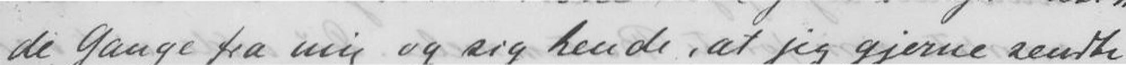inde Gange fra mig og sig hende, at jeg gjerne sendte
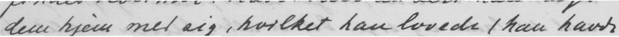dem hjem med sig, hvilket han lovede (han havde
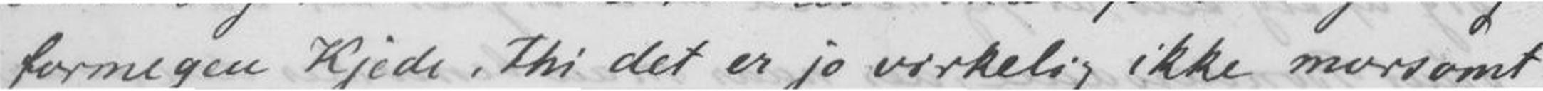formegen Kjede. Thi det er jo virkelig ikke morsomt
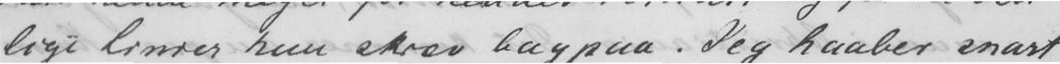lige linier hun skrev bagpaa. Jeg haaber snart
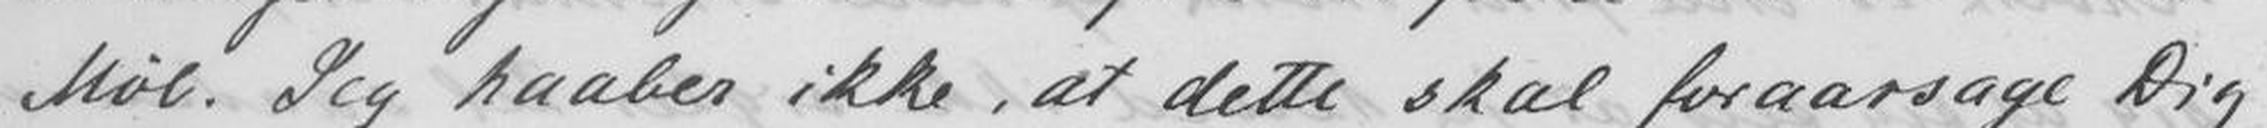Møl. Jeg haaber ikke, at dette skal foraarsage Dig
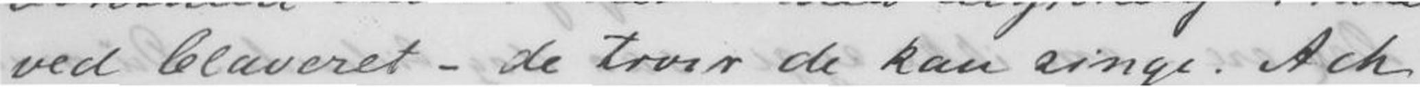ved Claveret - de troer de kan synge. Ach
